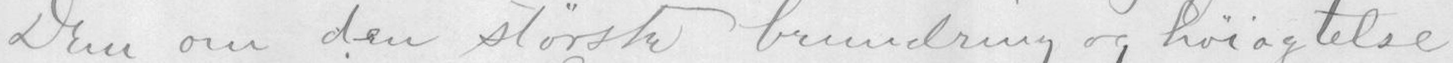Dem om den største beundring og høiagtelse
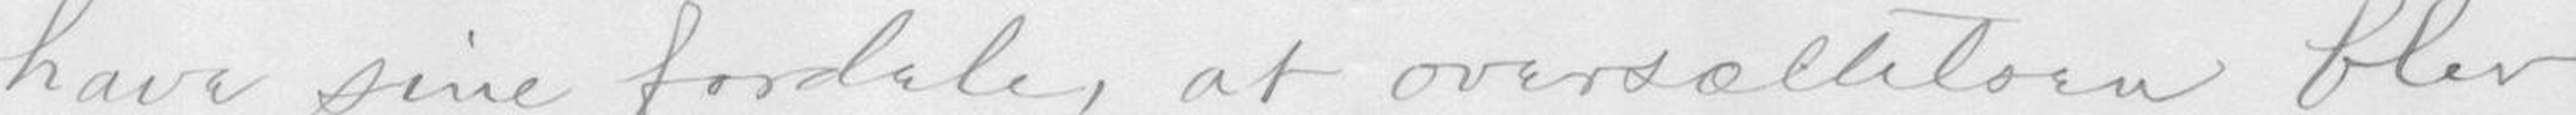have sine fordele, at oversættelsen blev
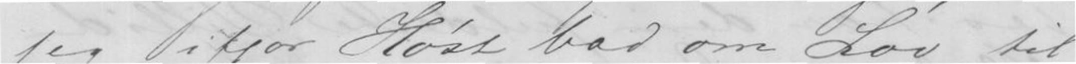jeg ifjor Høst bad om Lov til
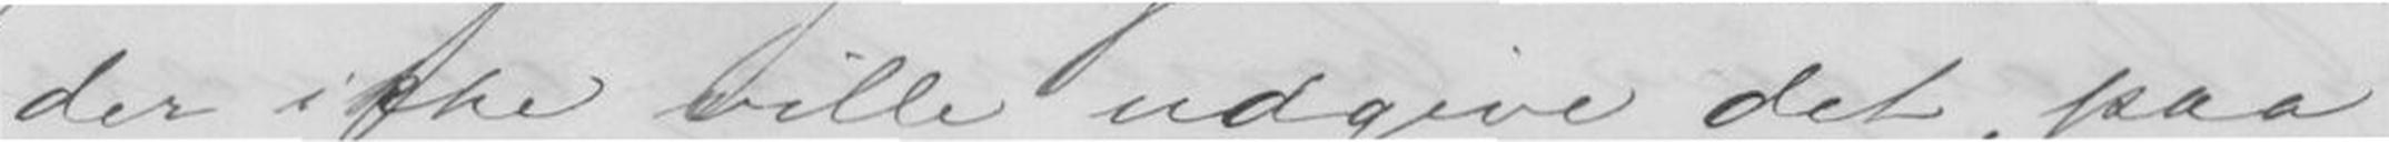der ikke ville udgive det, paa
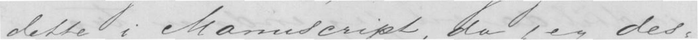dette i Manuscript, da jeg des-
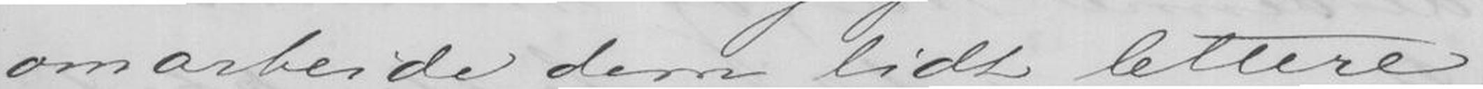omarbeide dem lidt lettere
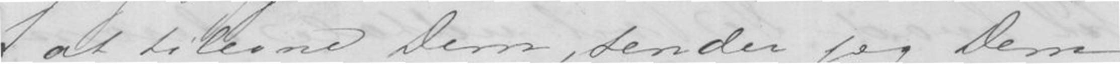at tilegne Dem, sender jeg Dem
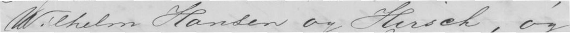Wilhelm Hansen og Hirsch, og
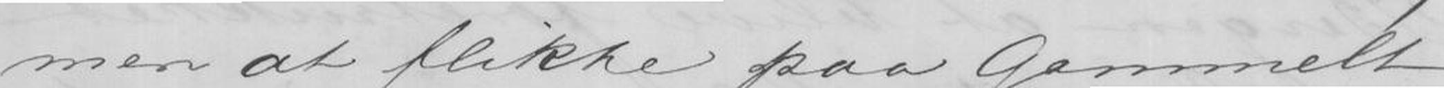men at flikke paa Gammelt
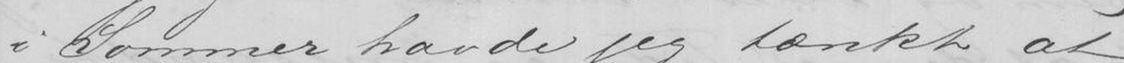i Sommer havde jeg tænkt at
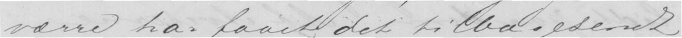værre har faaet det tilbagesendt
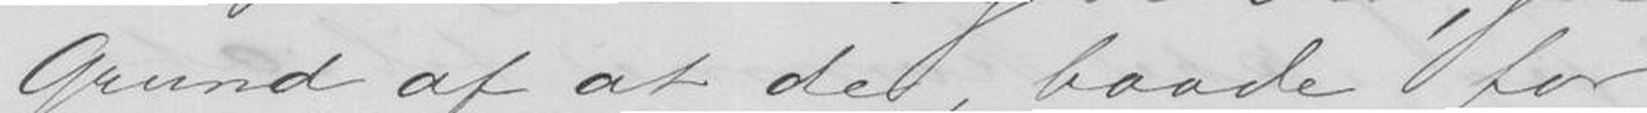Grund af at de, baade for
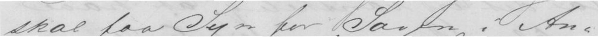skal faa Syn for Sagen i An-
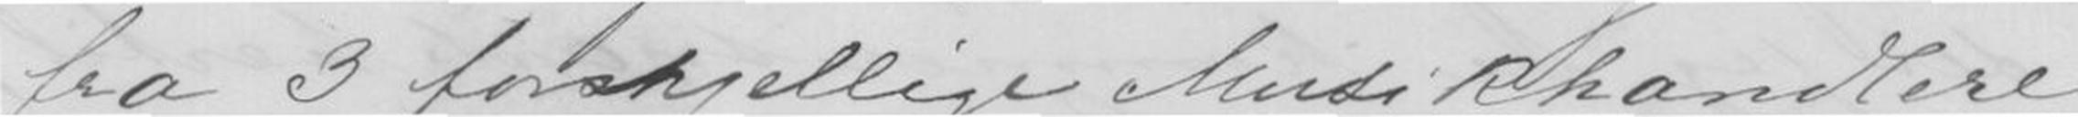fra 3 forskjellige Musikhandlere,
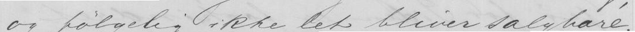og følgelig ikke let bliver salgbare.
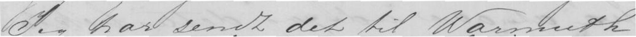Jeg har sendt det til Warmuth,
